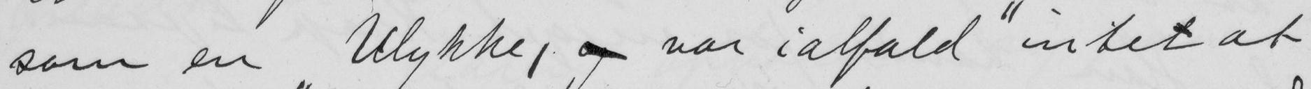som en Ulykke, og var ialfald "intet at
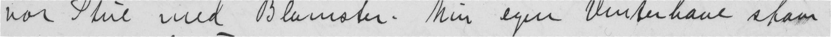vor Stue med Blomster. Min egen Vinterhave staar
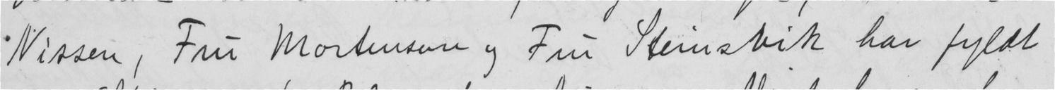Nissen, Fru Mortenson og Fru Steinsvik har fyldt
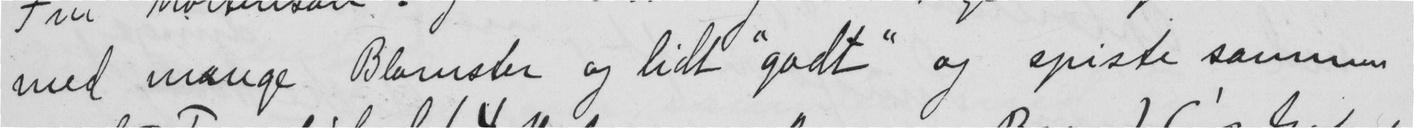med mange Blomster og lidt "godt" og spiste sammen
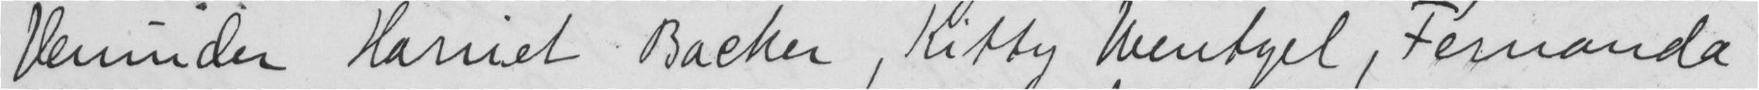Veninder Harriet Backer, Kitty Wentzel, Fernanda
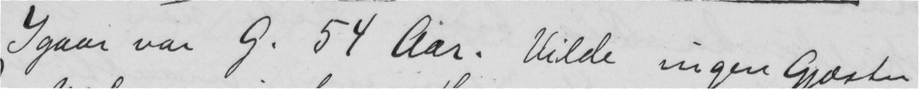Igaar var G. 54 Aar. Vilde ingen Gjæster
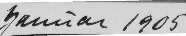Januar 1905
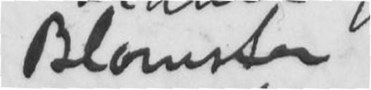Blomster
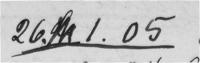26. 1.05.
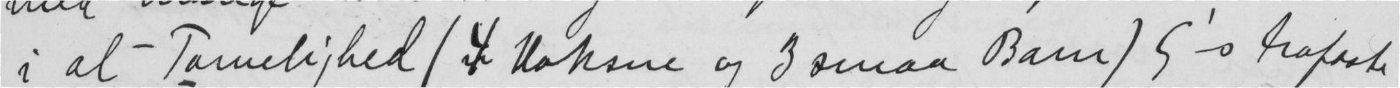i al-Tamelighed (4 Voksne og 3 smaa Barn) G' s trofaste
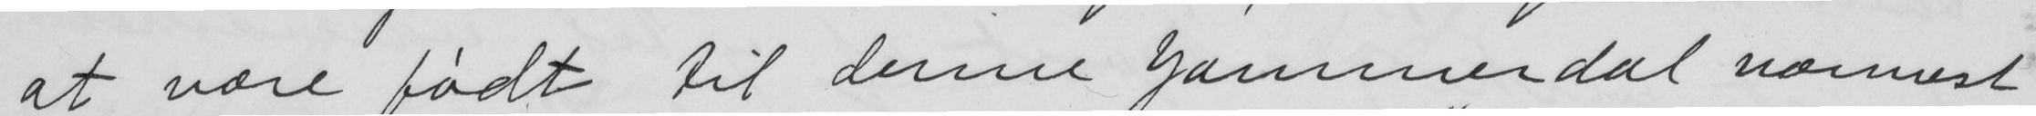at være født til denne Jammerdal nærmest
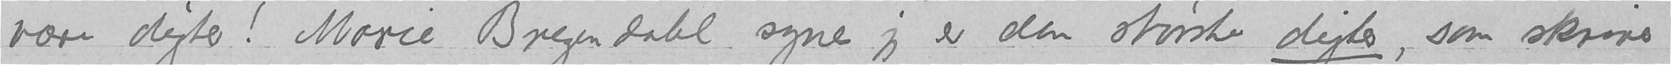være digter! Marie Bregendahl synes jeg er den største digter, som skriver
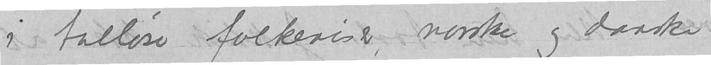i talløse folkeviser, norske og danske.
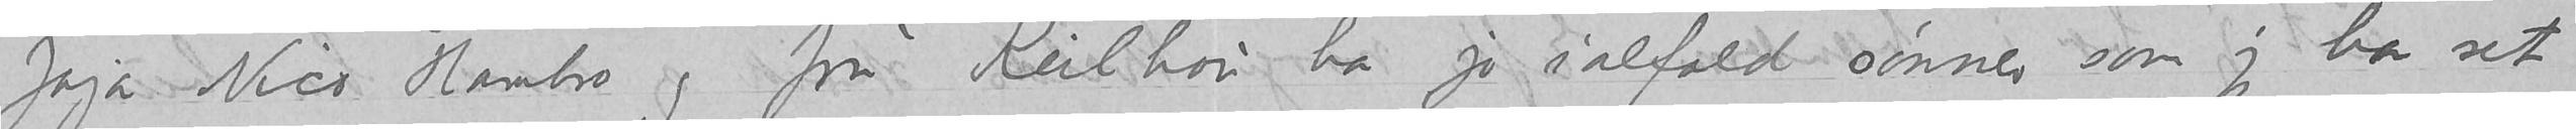Jaja Nico Hambro og fru Keilhau har jo ialfald sønner som jeg har set
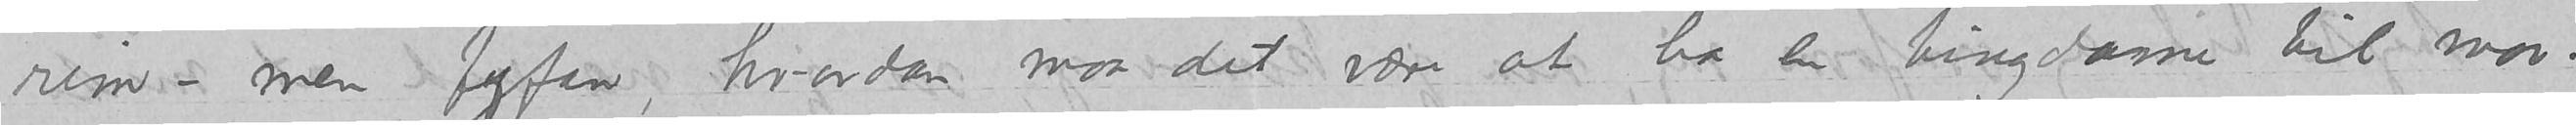rim – men fyfan, hvordan maa det være at ha en tingdame til mor
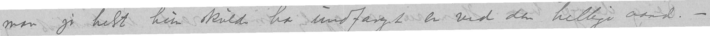man jo helst hun skulde ha undfanget en ved den hellige aand. –
